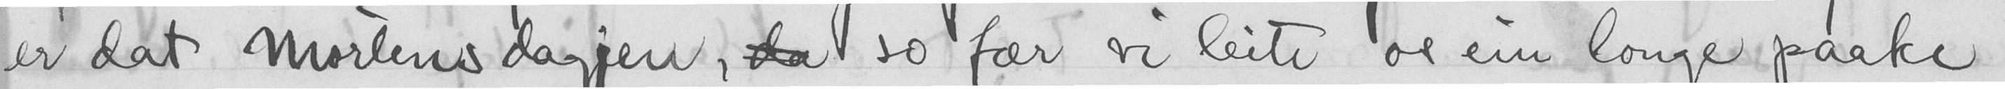er dat mortensdagjen,  so fær vi leite os ein longe paake
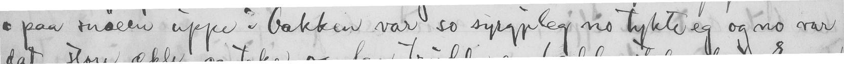paa snøeen uppe i bakken var jo syrgjeleg no tykte eg og no var
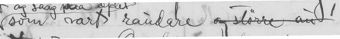som vart raudare
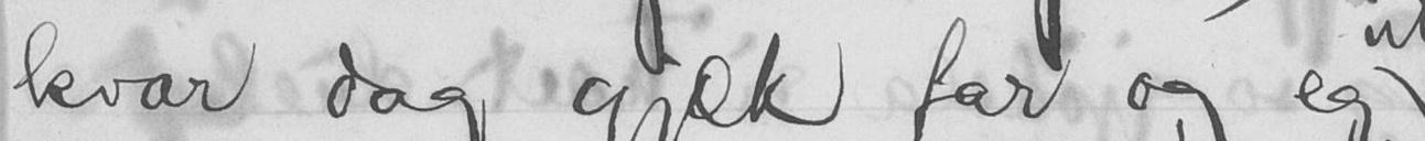hvar dag gjæk far og eg
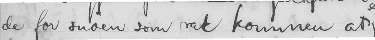de for snøen som var kommen at
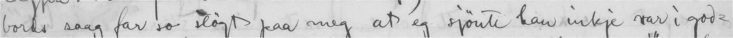bords saag far so sløgt paa meg at eg sjønte kan inkje var i god-
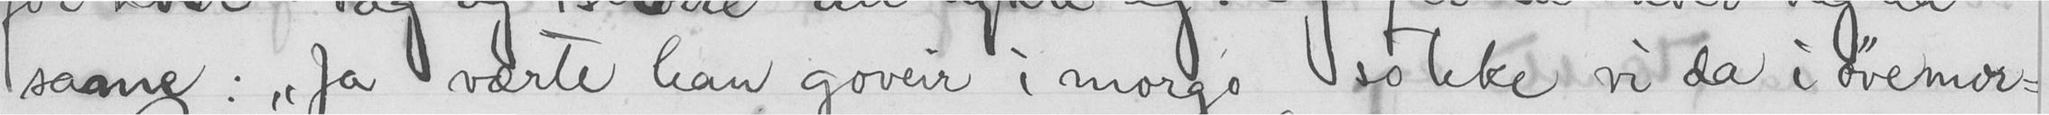saang: "Ja værte han goveir i morgo so teke vi da i øvemor-
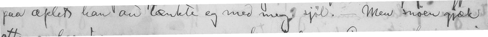paa æplet han an tænkte eg med meg sjøl. - Men snøen gjæk
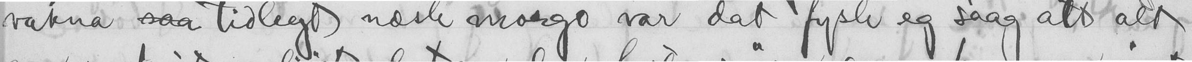vakna  tidligt næste morgo var dat fyste eg saag att alt
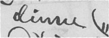dinne
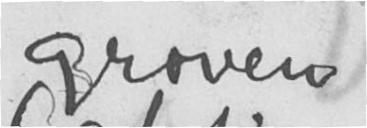groven
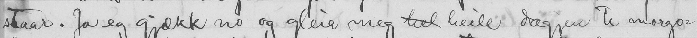staar. Ja eg gjækk no og gleia meg  heile dagjen te morgo-
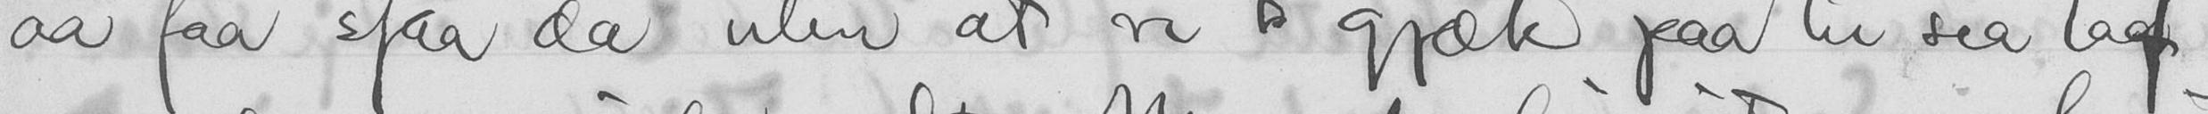aa faa sjaa da uten at vi to gjæk paa hi sia taa
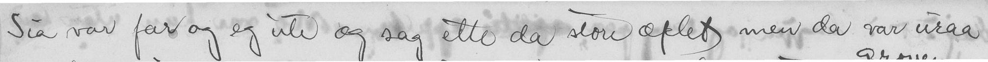Sia var far og eg ute og sag ette da store æplet men, da var uraa
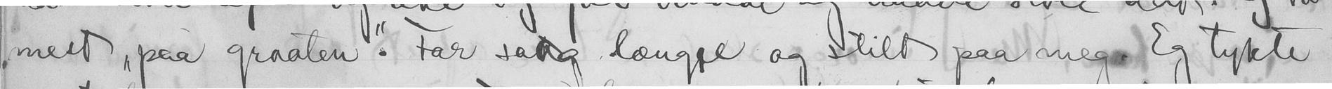mest "paa graaten". For satt længe og stilt paa meg. Eg tykte
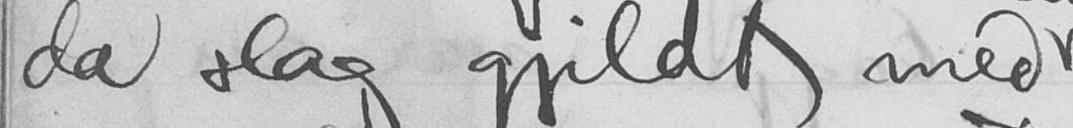da slag gjildt med
